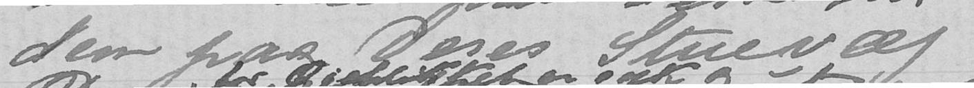dem paa Deres Stuevæg.
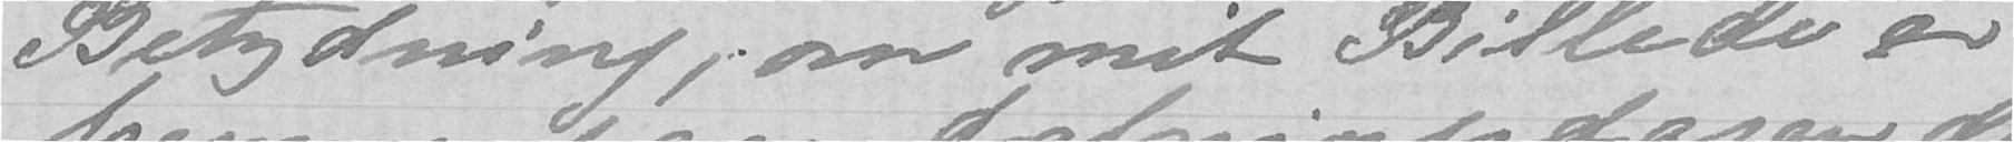Betydning, om mit Billede er
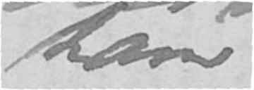ham
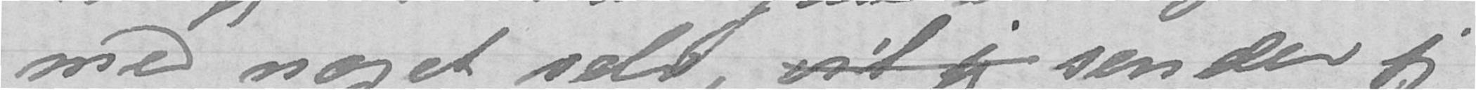med noget selv, vil jeg sender jeg
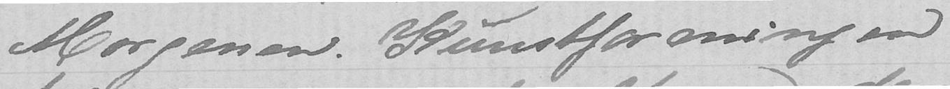Morgenen. Kunstforeningen
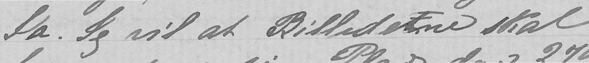Ja. Jeg vil at Billederne skal
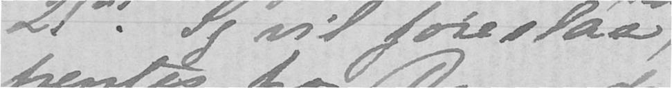21de. Jeg vil foreslaa
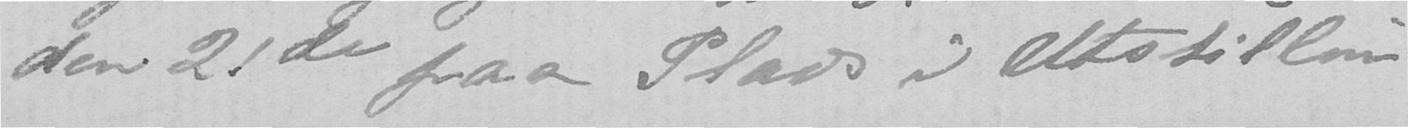den 21de paa Plads i Utstillin-
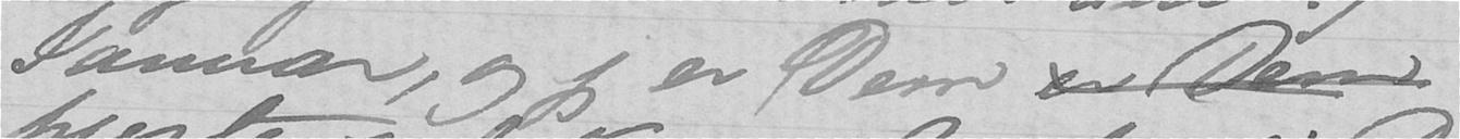Januar, og jeg er Dem er Dem
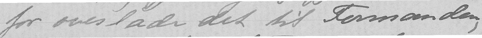for overlade det til Formanden,
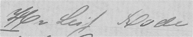Hr Leif Rode
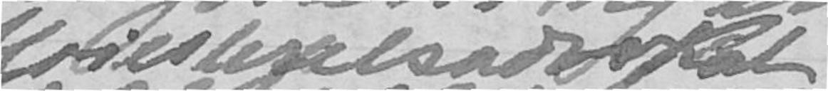Høiesteretsadvokat
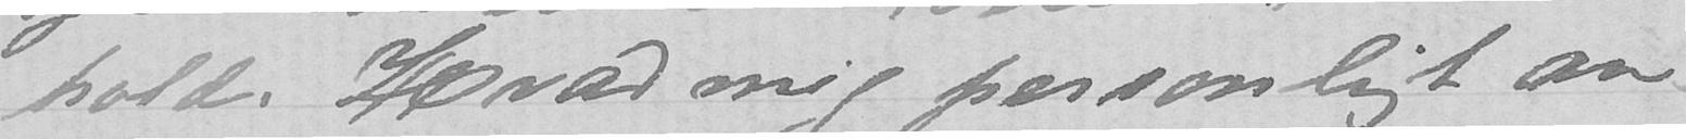hold. Hvad mig personligt an-
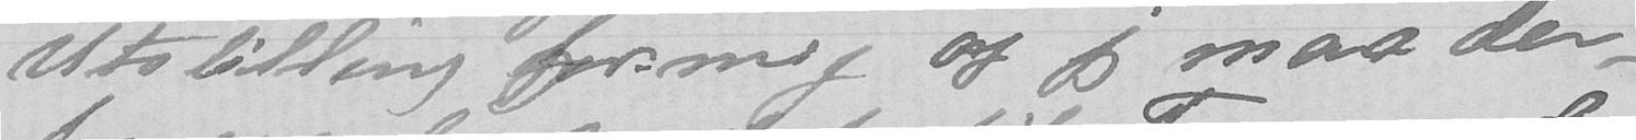Utstilling for mig og jeg maa der-
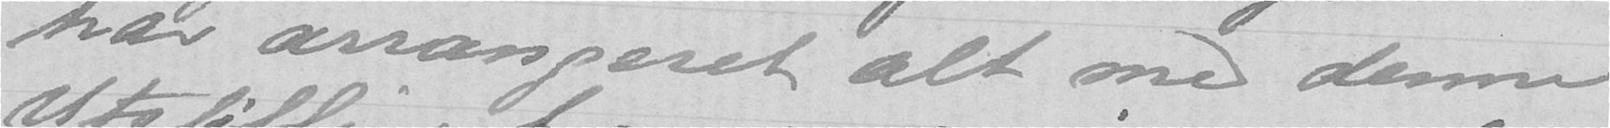har arrangeret alt med denne
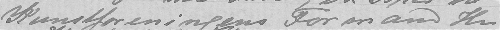Kunstforeningens Formand Hr
